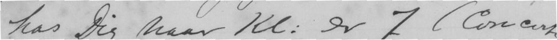hos Dig naar Kl. er 7 (Concerten
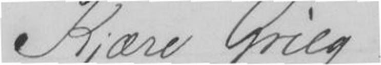Kjære Grieg!
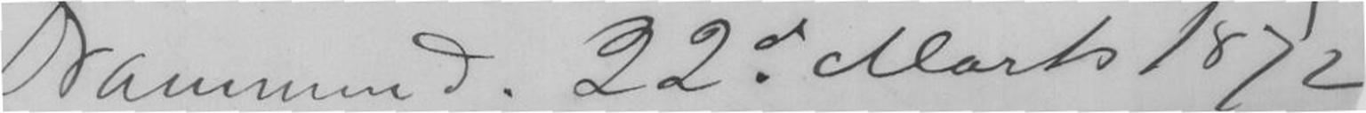Drammen d. 22d Marts 1872
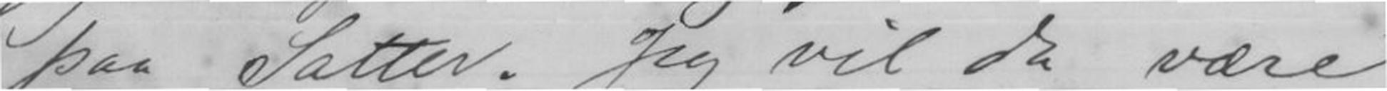paa Satter. Jeg vil da være
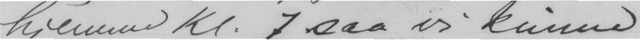hjemme Kl. 7 saa vi kunne
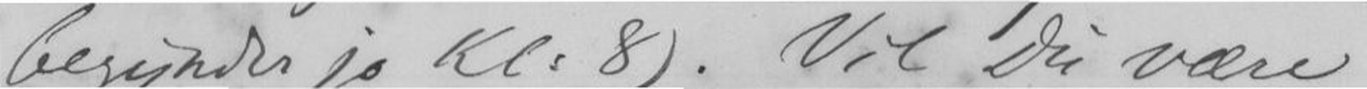begynder jo Kl. 8). Vil Du være
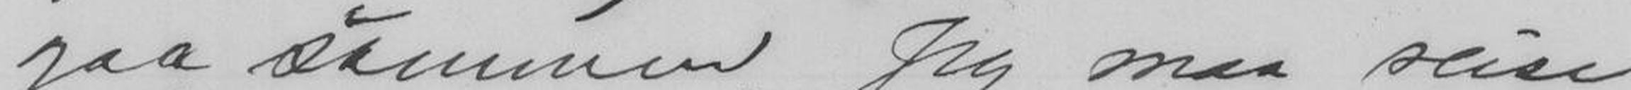gaa Sammen. Jeg maa reise
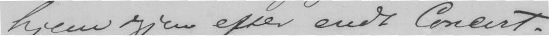hjem igjen efter endt Concert.
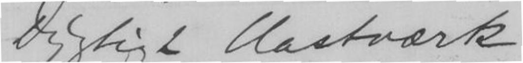Dygtigt Hastværk
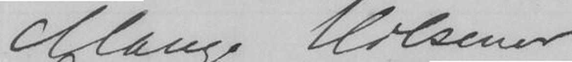Mange Hilsener!
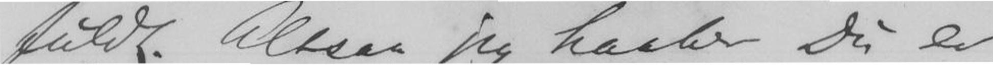fuldt. Altsaa jeg haaber Du er
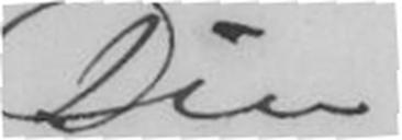Din
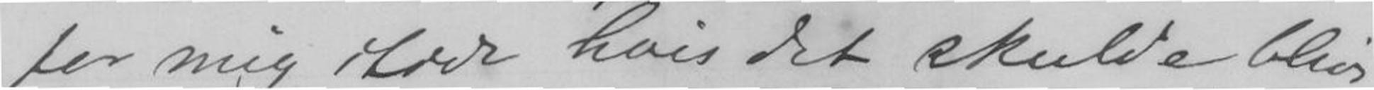for mig itide hvis det skulde blive
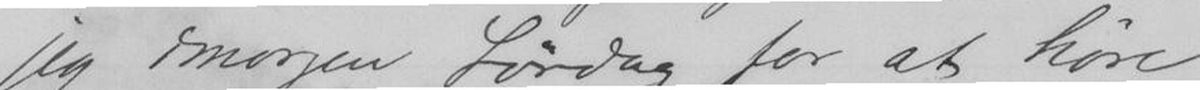jeg imorgen Lørdag for at høre
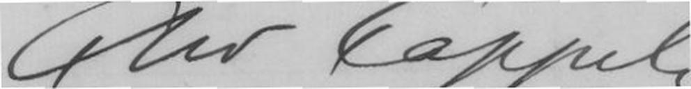Chr. Cappelen
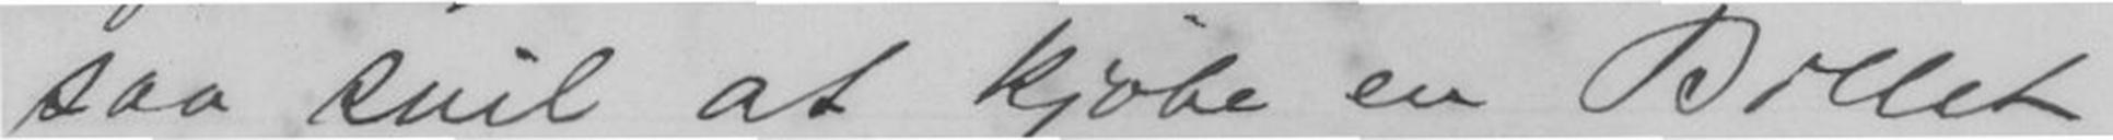saa snil at kjøbe en Billet
